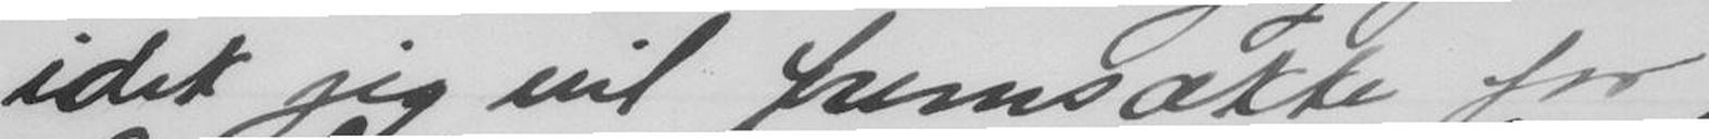idet jeg vil fremsætte for
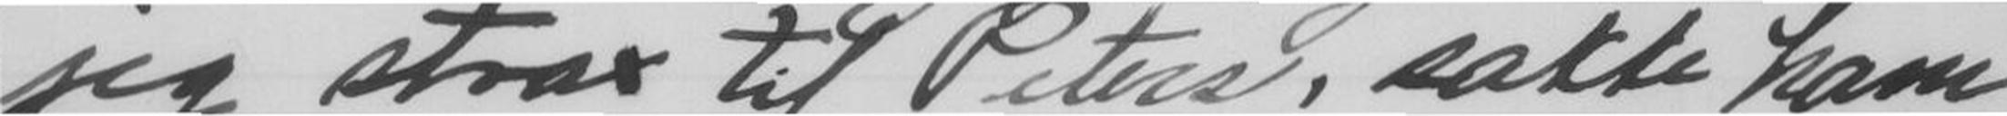jeg strax til Peters, satte ham
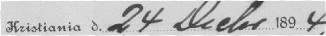Kristiania d. 24 Decbr 1894.
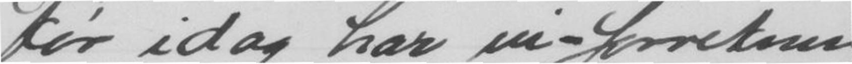Før idag har vi-forretningen
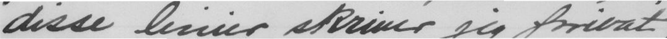disse linier skriver jeg privat,
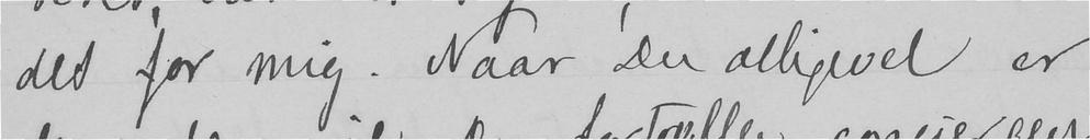det for mig. Naar Du alligevel er
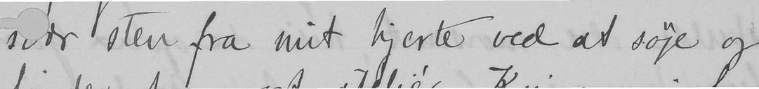svær sten fra mit hjerte ved at søge og
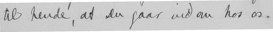til hende, at Du gaar indom hos os.
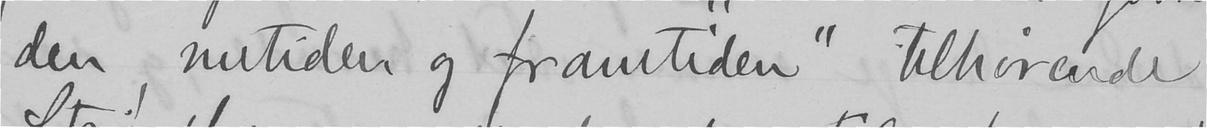den, nutiden og framtiden" tilhørende
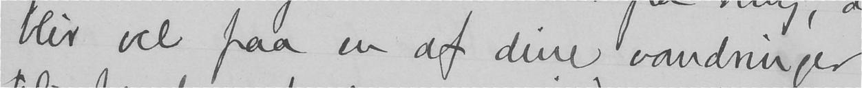blir vel paa en af dine vandringer
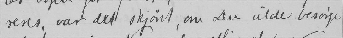reres var det skjønt, om Du vilde besørge
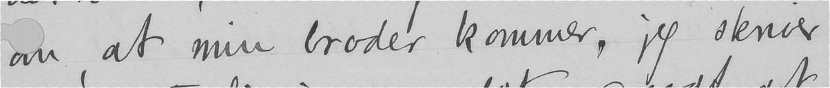om, at min broder kommer, jeg skriver
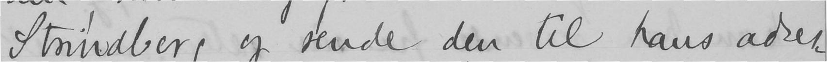Strindberg og sende den til hans adres-
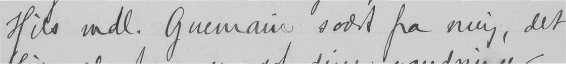Hils mdl. Guemain svært fra mig, det
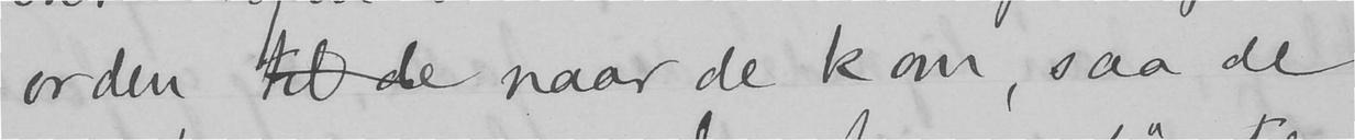orden til de naar de kom, saa de
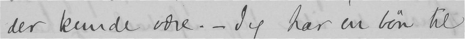der kunde være. - Jeg har en bøn til
